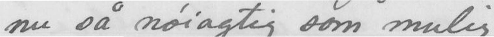nu så nøiagtig som mulig
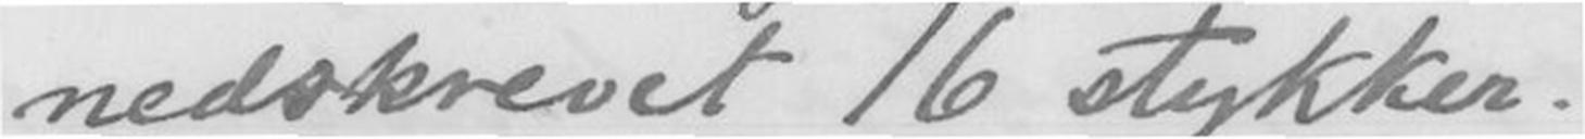nedskrevet 16 stykker.
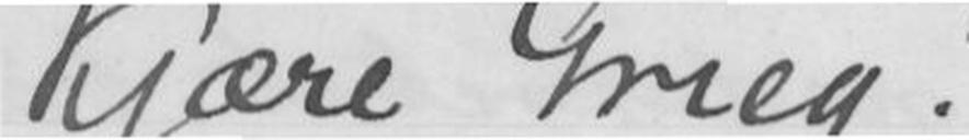Kjære Grieg!
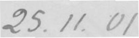25.11.01
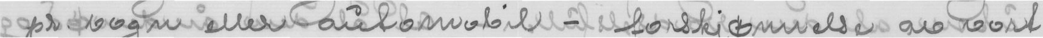pr. vogn eller automobil - forskjønnelse av vort
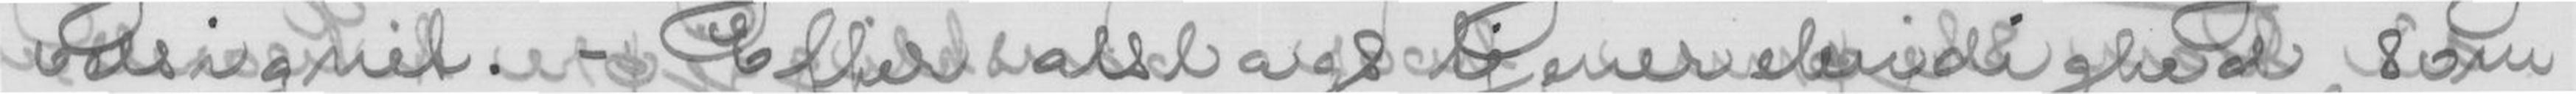velsignet. - Efter alslags tjenerelendighed, som
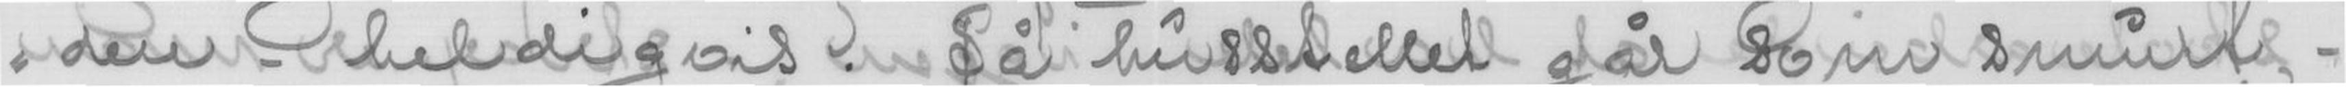den - heldigvis! Så husstellet går som smurt, -
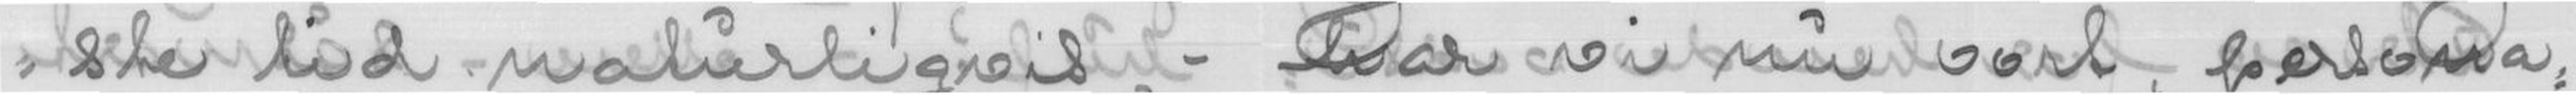ste tid naturligvis, - har vi nu vort persona-
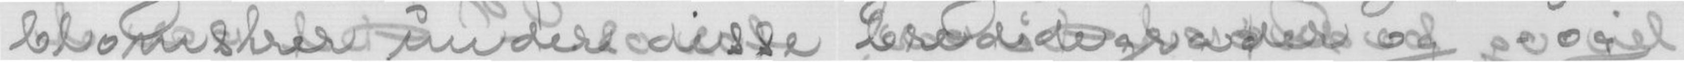blomstrer under disse breddegrader og, - og
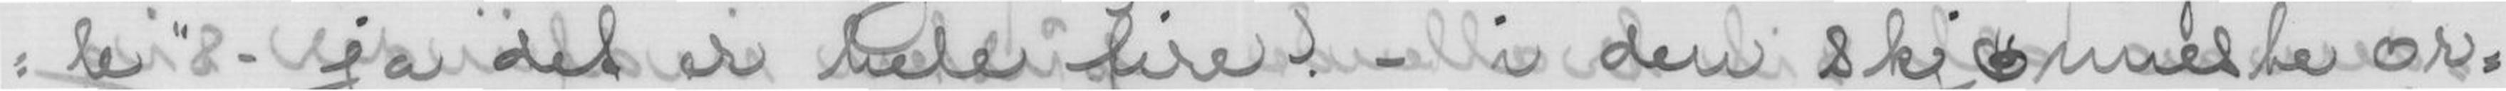le - ja det er hele fire! - i den skjønneste or-
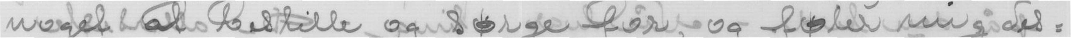noget at bestille og sørge for, og føler mig des-
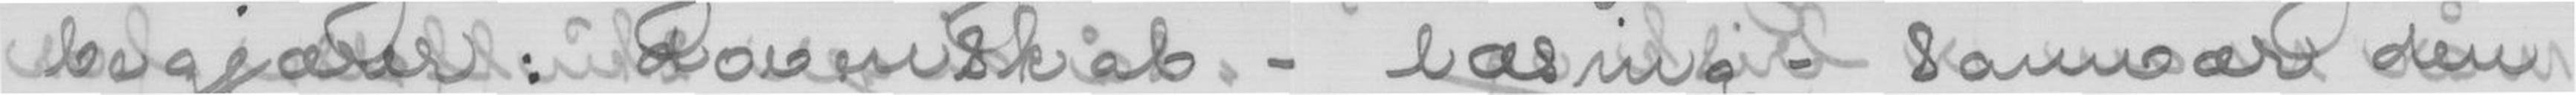begjærer: dovenskab - læsning - samvær den
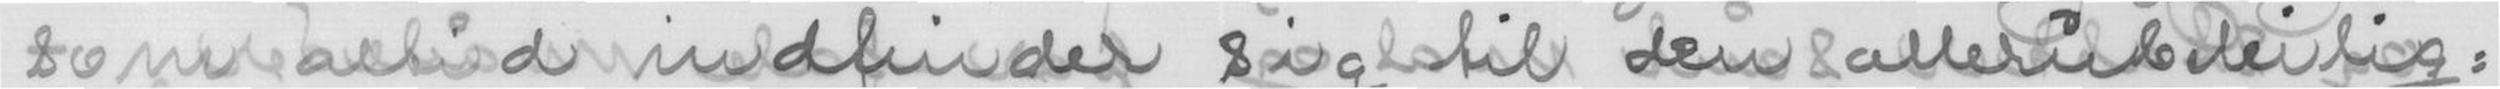som altid indfinder sig til den allerubeleilig-
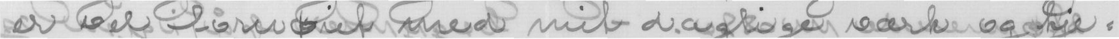er vel fornøiet med mit daglige værk og kje-
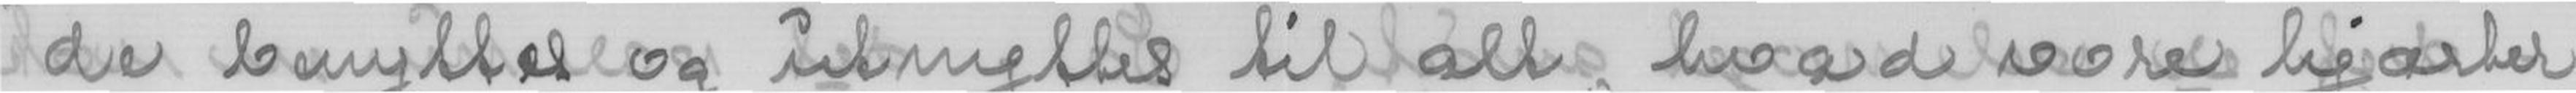de benyttes og utnyttes til alt hvad vore hjærter
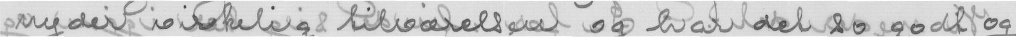nyder virkelig tilværelsen og har det so godt og
